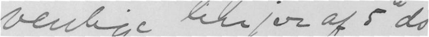venlige linjer af 5 ds.
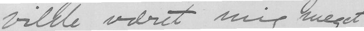vilde været mig meget
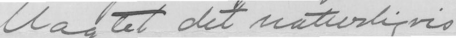Uagtet det naturligvis
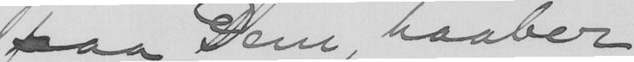paa Dem, haaber
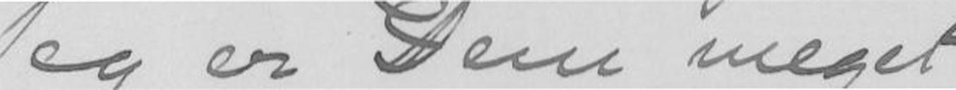Jeg er Dem meget
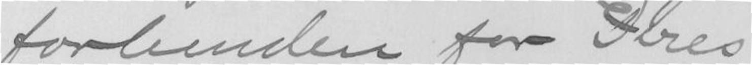forbunden for Deres
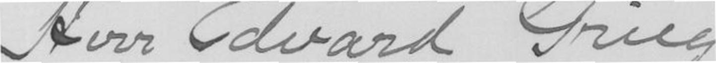Herr Edvard Grieg.
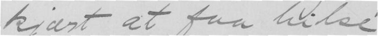kjært at faa hilse
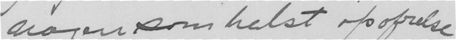nogensomhelst opofrelse
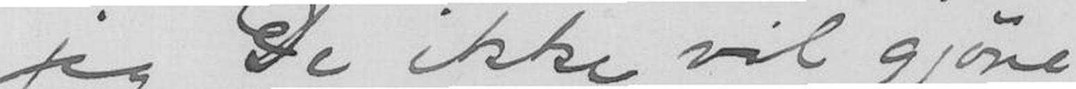jeg De ikke vil gjøre
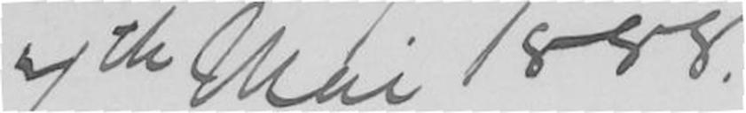7th Mai 1888.
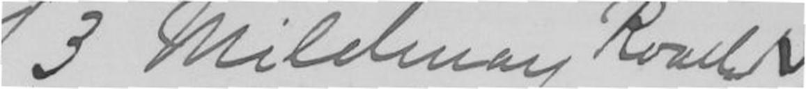93 Mildway Road N.
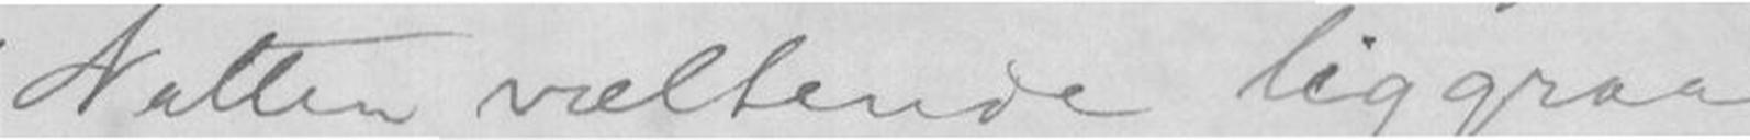Natten væltende liggraa
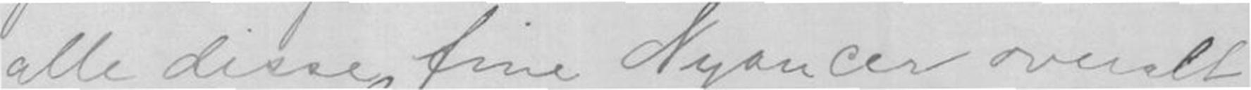alle disse fine Nyancer overalt
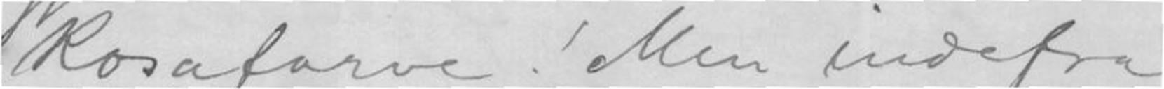Rosafarve! Men indefra
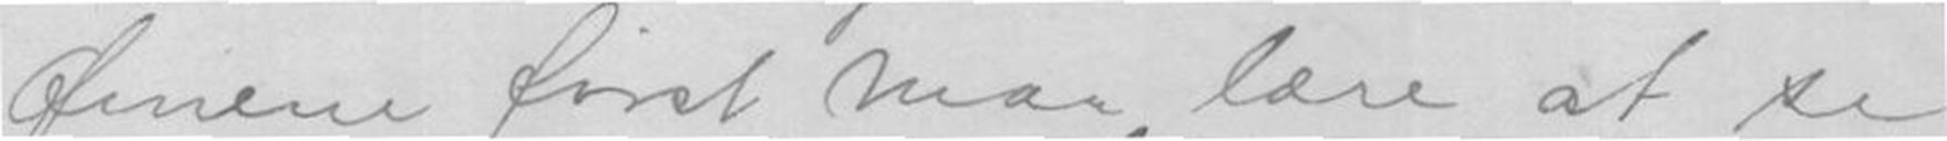Øinene først maa lære at se
